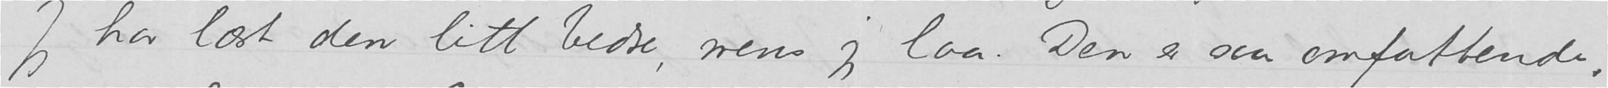Jeg har læst den litt bedre, mens jeg laa. Den er saa omfattende,
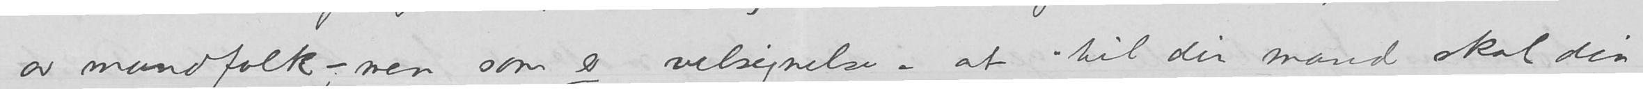av mandfolk – men som er velsignelse – at «til din mand skal din
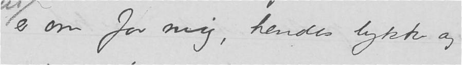er om for mig, hendes lykke og
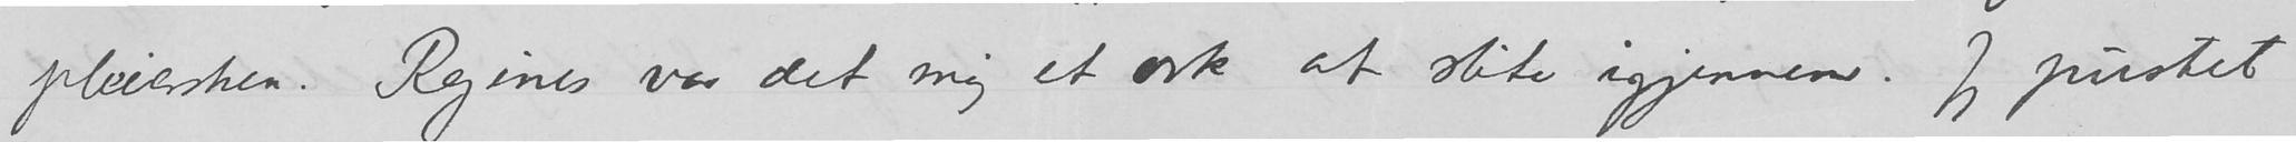pleiersken. Regines var det mig et ork at slite igjennem. Jeg pustet
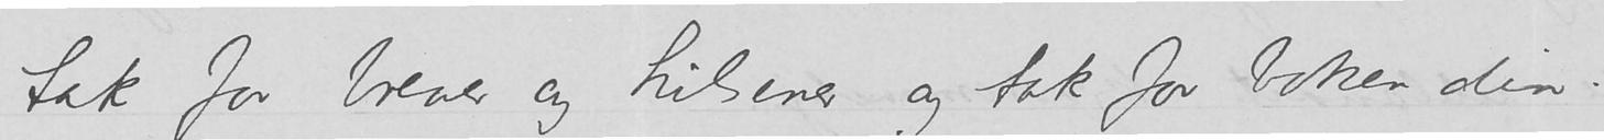tak for brever og hilsener og tak for boken din.
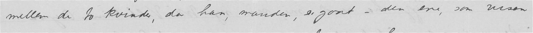mellem de to kvinder, da han, manden, er gaat – den ene, som vissen
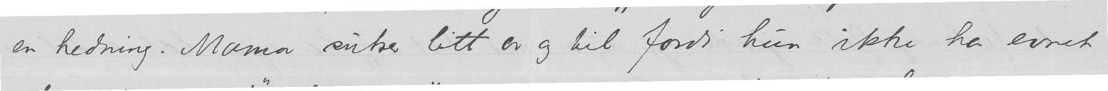en hedning. Mama sutrer litt av og til fordi hun ikke ha evnet
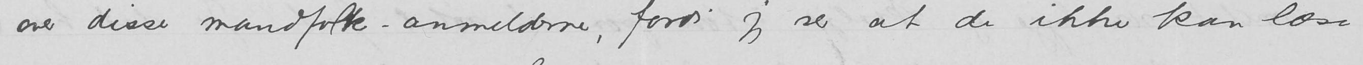over disse mandfolk–anmelderne, fordi jeg ser at de ikke kan lære
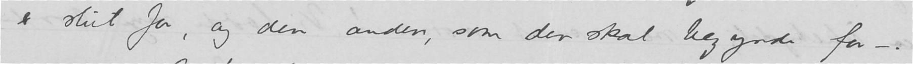er slut for, og den anden, som den skal begynde for –.
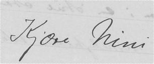Kjære Nini,
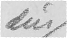Din
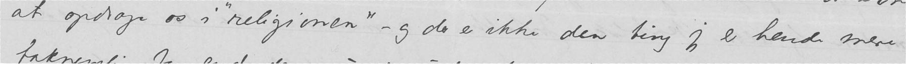at opdrage os i «religionen» –- og der er ikke den ting jeg er hende mere
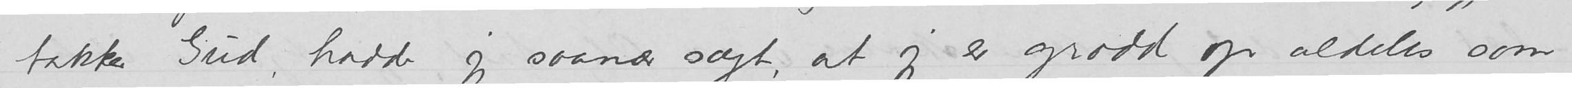takker Gud, hadde jeg saanær sagt, at jeg er grodd op aldeles som
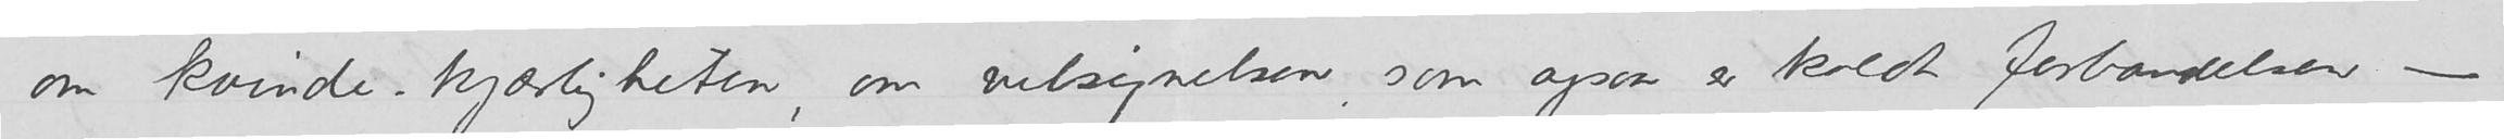om kvinde-kjærligheten, om velsignelsen, som ogsaa er kaldt forbandelsen –
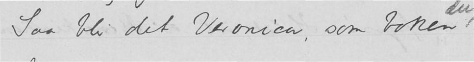Saa blir det Veronica, som boken
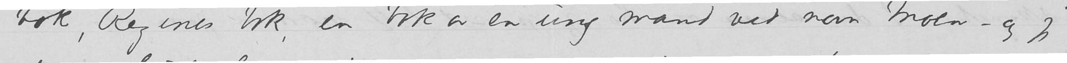bok, Regines bok, en bok av en ung mand ved navn Moen – og jeg
